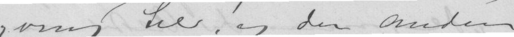ving til, og den Anden
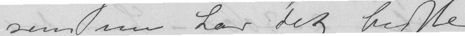som nu har det bedste
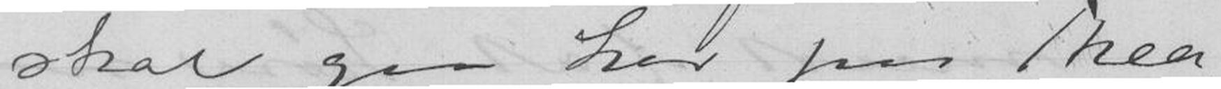skal gaa her paa Thea-
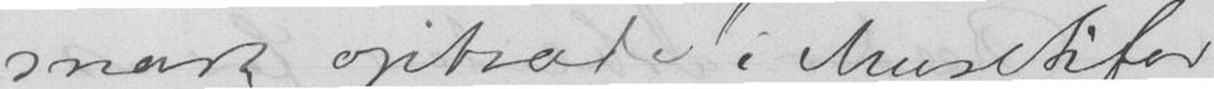snart optræde i Musikfor-
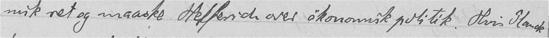nisk ret og maaske Helferich over økonomisk politik. Hvis Planck
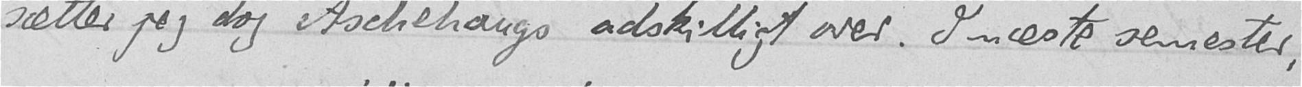sætter jeg dog Aschehougs adskilligt over. I næste semester,
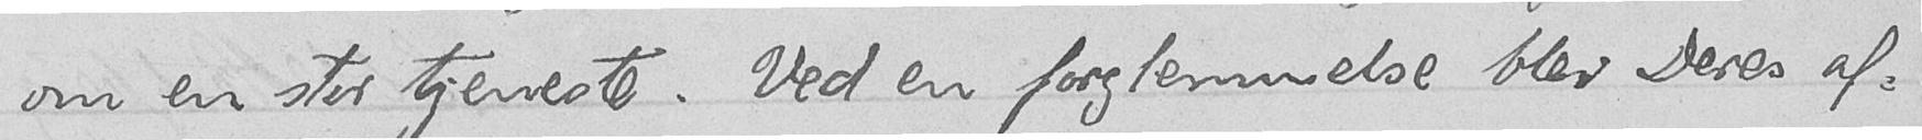om en stor tjeneste. Ved en forglemmelse blev Deres af-
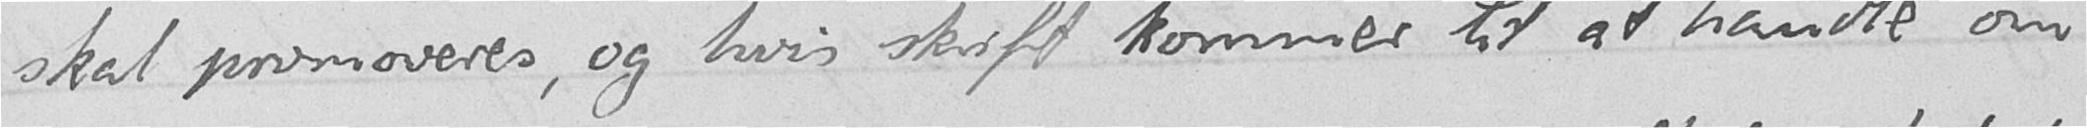skal promoveres, og hvis skrift kommer til at handle om
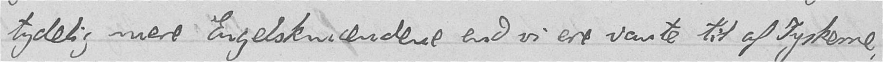tydelig mere Engelskmændene end vi ere vante til af Tyskerne,
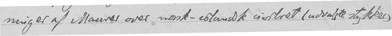ninger af Maurer over norsk-islandsk civilret (udvalgte stykker)
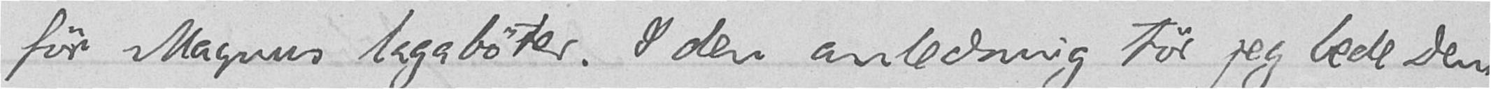før Magnus lagabøter. I den anledning tør jeg bede Dem
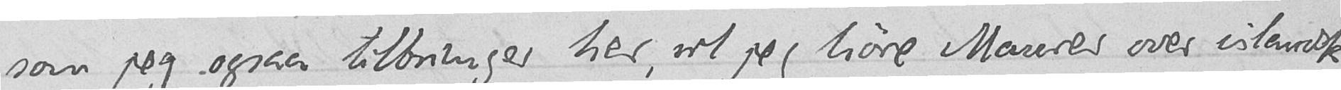som jeg ogsaa tilbringer her, vil jeg høre Maurer over islandsk
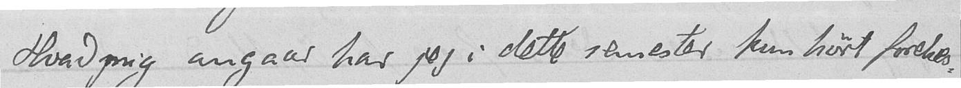Hvad mig angaar har jeg i dette semester kun hørt forelæs-
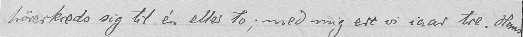hørerkreds sig til én eller to; med mig ere vi iaar tre. Hans
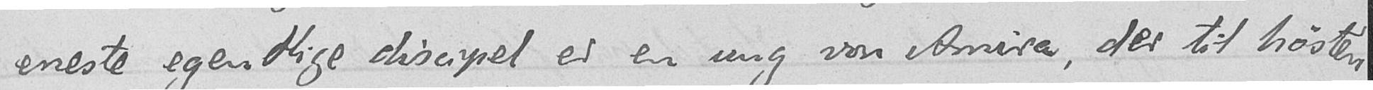eneste egentlige discipel er en ung von Amira, der til høsten
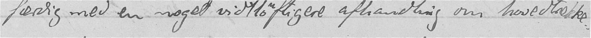færdig med en noget vidtløftigere afhandling om "hovedtrække-
